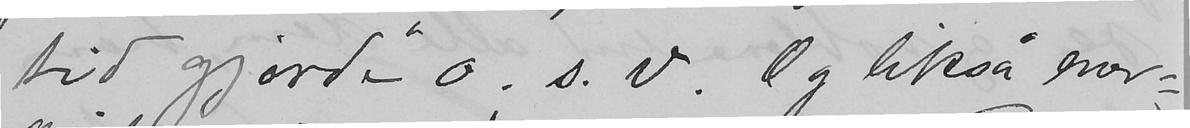tid gjorde" o.s.v. Og likså ener-
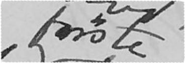første
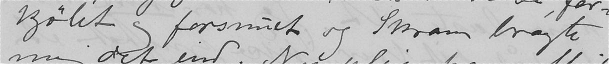kjølet og forsnuet og Skram bragte
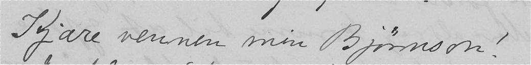Kjære vennen min Bjørnson!
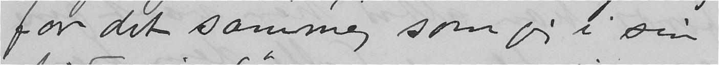for det samme, som jeg i sin
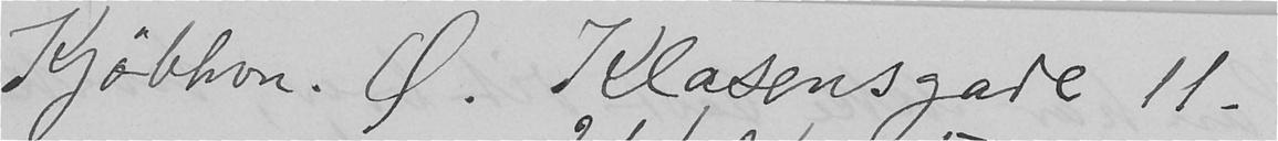Kjøbhvn. Ø. Klasensgade 11
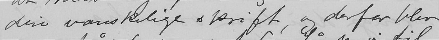din vanskelige skrift, og derfor blev
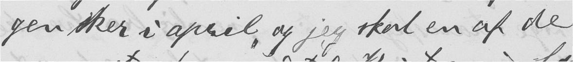gen sker i april, og jeg skal en af de
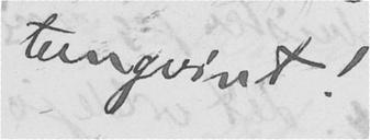tungvint!
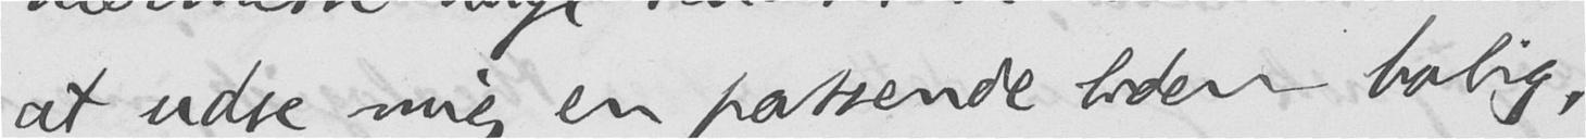at udse mig en passende liden bolig,
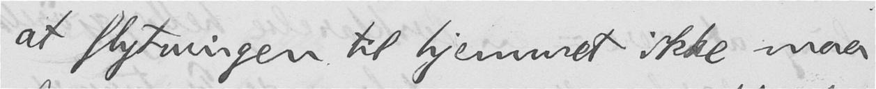at flytningen til hjemmet ikke maa
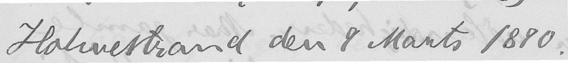Holmestrand den 9 Marts 1890.
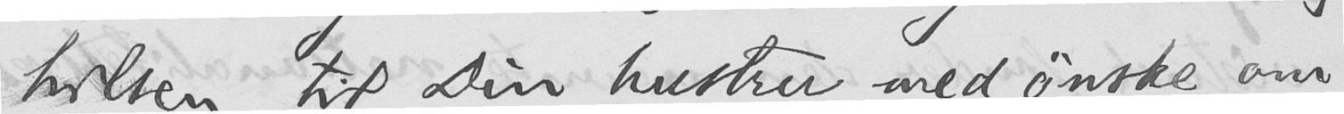hilsen til Din hustru med ønske om
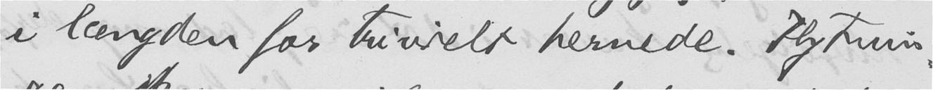i længden for trivielt hernede. Flytnin-
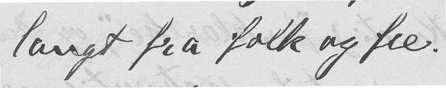langt fra folk og fæ.
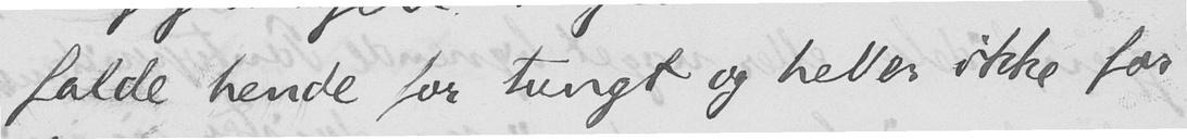falde hende for tungt og heller ikke for
